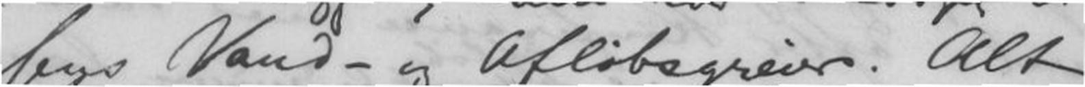bys Vand - og Afløbsgreier. Alt
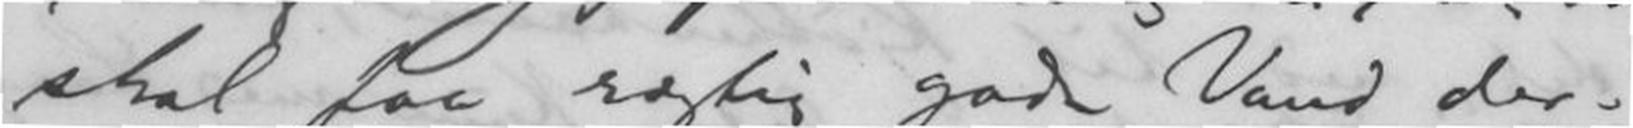skal faa rigtig godt Vand der-
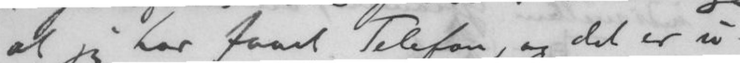at jeg har faaet Telefon, og det er u-
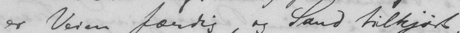er veien færdig, og Sand tilkjørt,
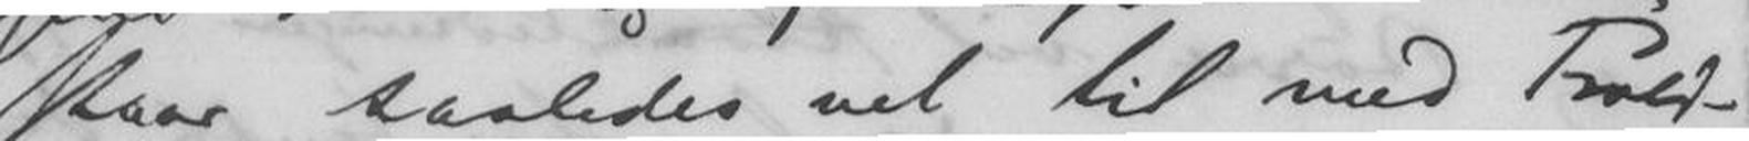staar saaledes vel til med Trold-
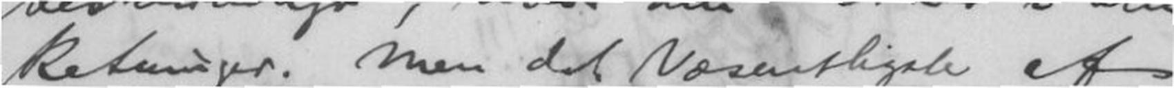Retninger. Men det Væsentligste af
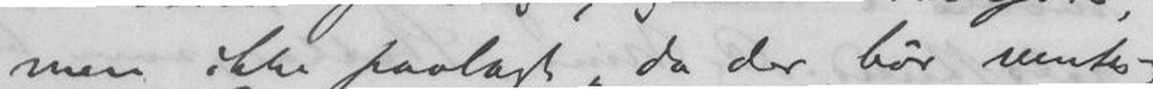men ikke paalagt, da der bør ventes
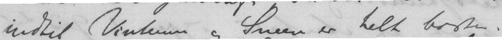intil Vinteren og Sneen er helt borte.
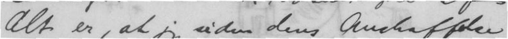Alt er, at jeg siden dens Anskaffelse
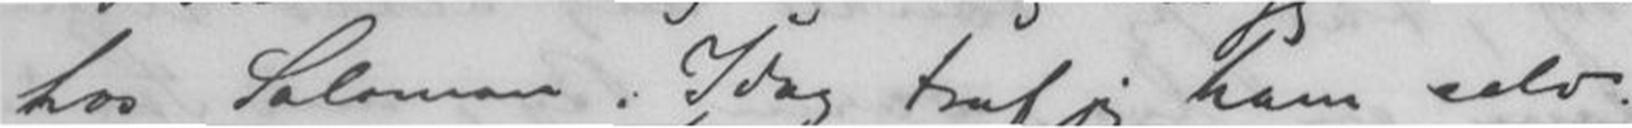hos Salomon. Idag traf jeg ham selv.
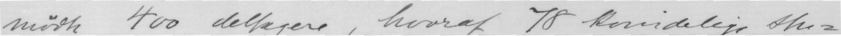mødte 400 deltagere, hvoraf 78 kvindelige stu-
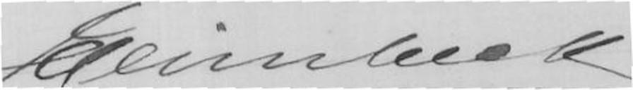J. Heimbeck.
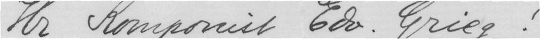Hr komponist Edv. Grieg!
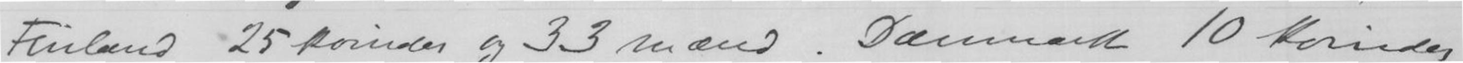Finland 25 Kvinder og 33 Mænd. Danmark 10 Kvinder
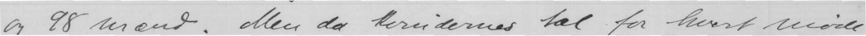og 98 Mænd. Men da kvindernes tal for hvert møde
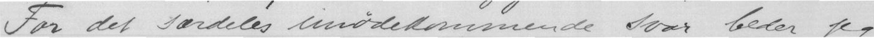For det særdeles imødekommende svar beder jeg
